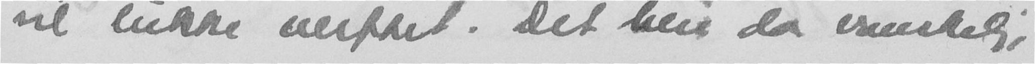vil lukke verftet. Det blir da vanskelig,
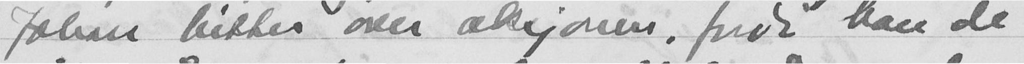Johan bitter over aksjonen, fordi han de
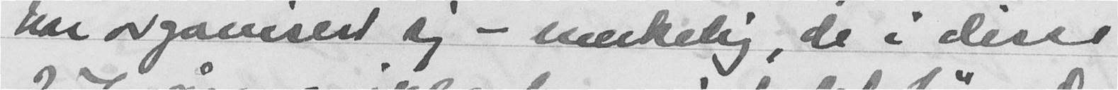har organisert sig - merkelig, de i disse
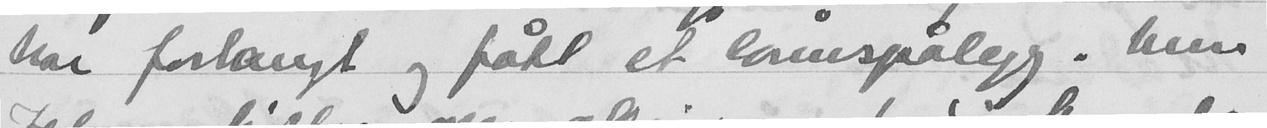har forlangt og fått et lønnspålegg. Men
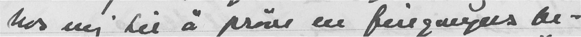hos mig til å prøve en feilgangers be-
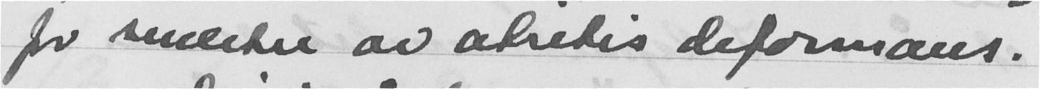for smerten av atritis deformans.
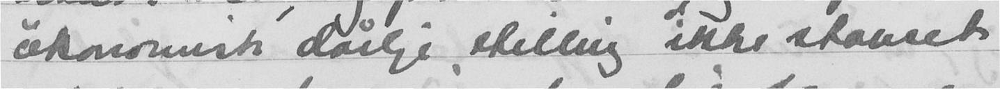økonomisk dårlige stilling ikke stanset
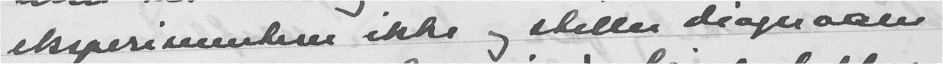eksperimenterer ikke og stiller diagnosen
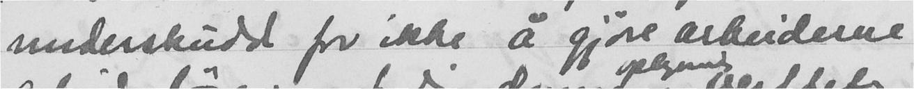underskudd for ikke å gjøre arbeiderne
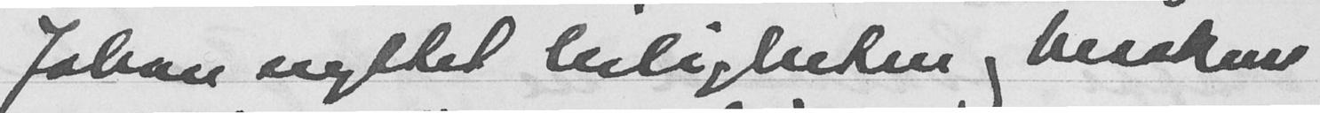Johan nyttet leiligheten og besøkene
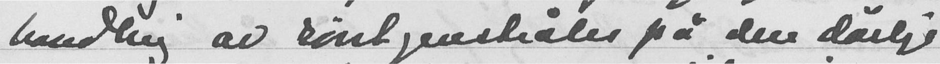bandling av røntgenstråler på den dårlige
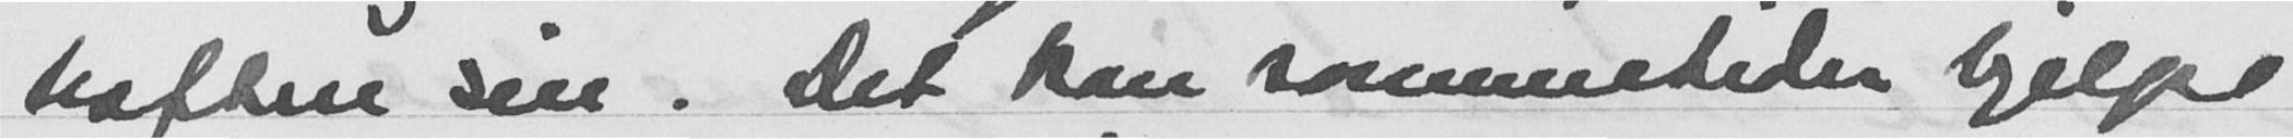hoften sin. Det kan sommetider hjelpe
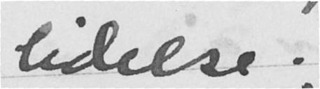lidelse.
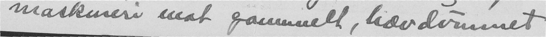maskineri mot gammelt, trævdommet
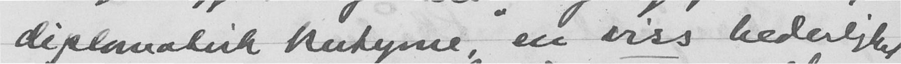diplomatisk kntyrne en viss hederlighet
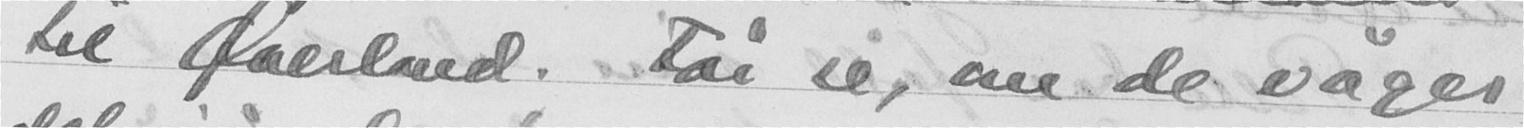til Øverland. Får se, om de våger
